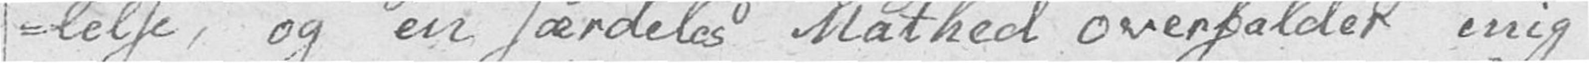-lelse, og en særdeles Mathed overfalder mig
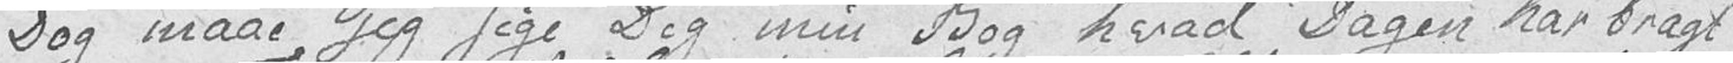Dog maae jeg sige Dig min Bog hvad Dagen har bragt
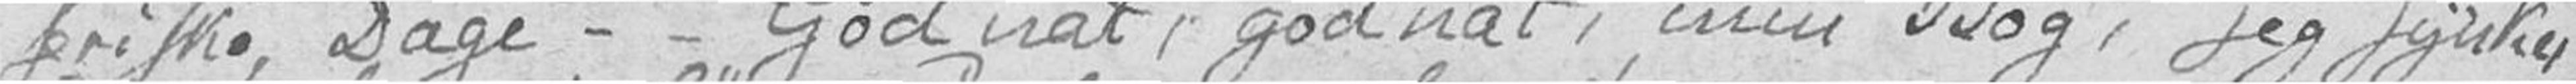friske Dage – – God nat, god nat, min Bog, jeg synker
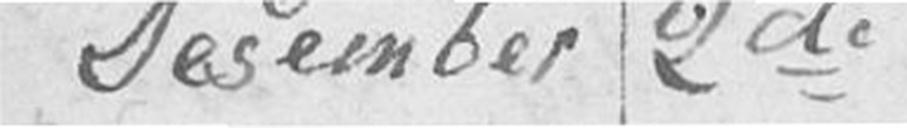Desember 2de
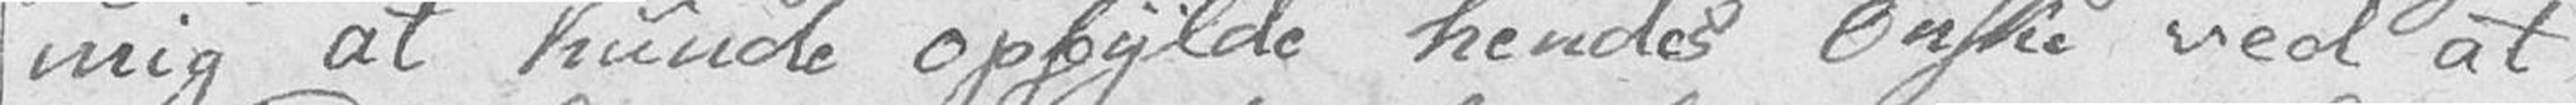mig at kunde opfylde hendes Ønske ved at
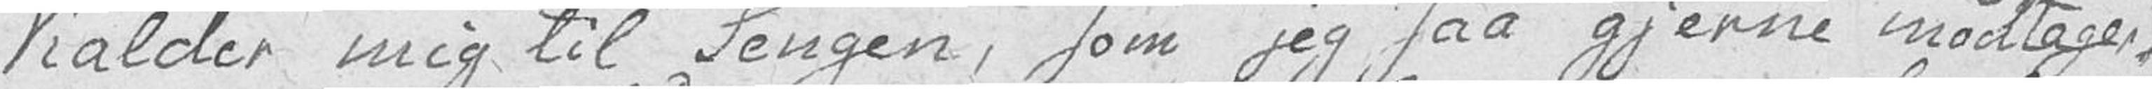kalder mig til Sengen, som jeg saa gjerne modtager.
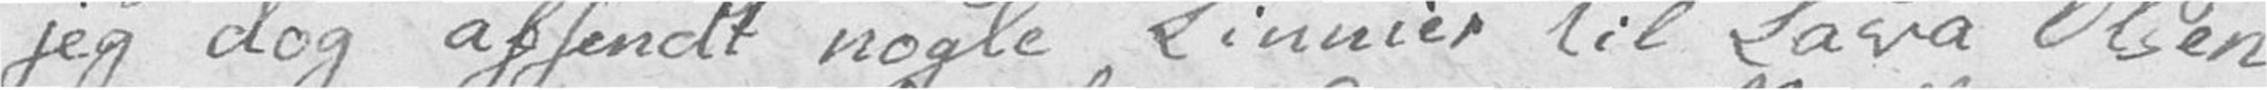jeg dog afsendt nogle Linnier til Lava Olsen
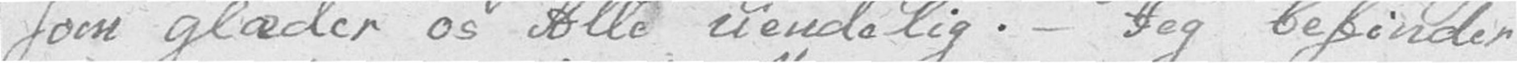som glæder os Alle uendelig. – Jeg befinder
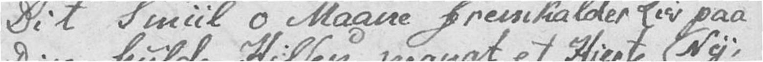Dit Smiil o Maane fremkalder Liv paa Ny,
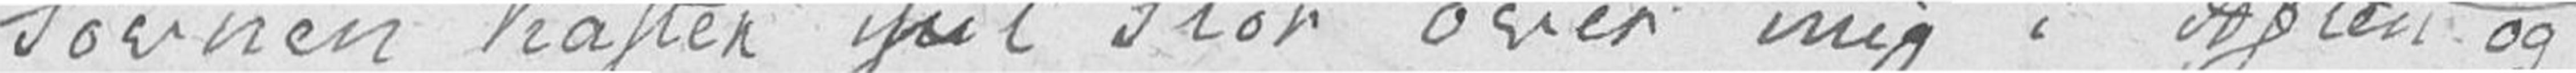Søvnen kaster sit Slør over mig i Aften og
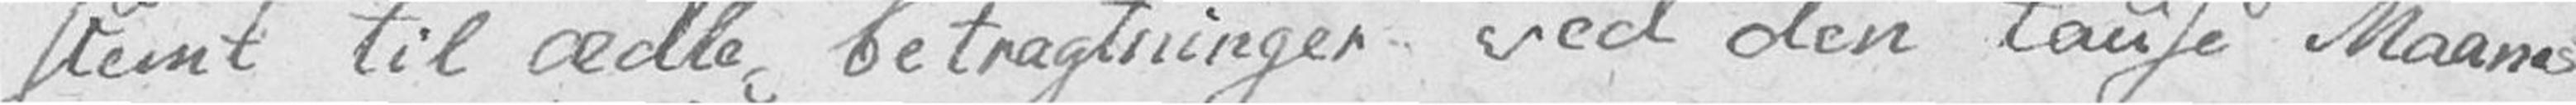stemt til ædle betragtninger ved den tause Maanes
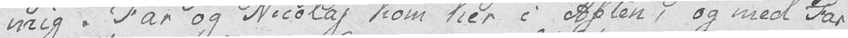mig. Far og Nicolaj kom her i Aften, og med Far
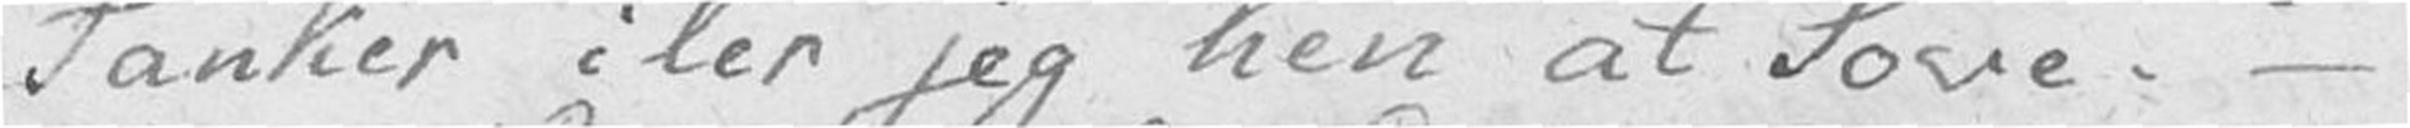Tanker iler jeg hen at Sove. –
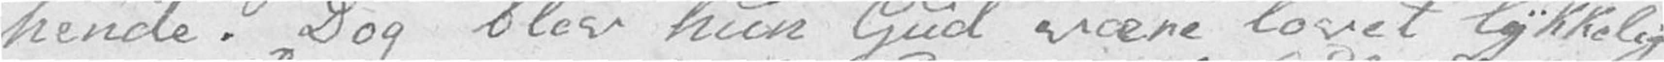hende. Dog blev hun Gud være lovet Lykkelig
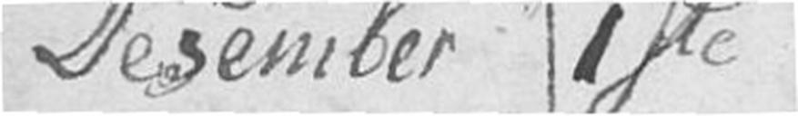Desember 1ste
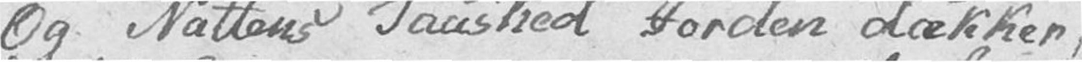Og Nattens Taushed Jorden dækker,
